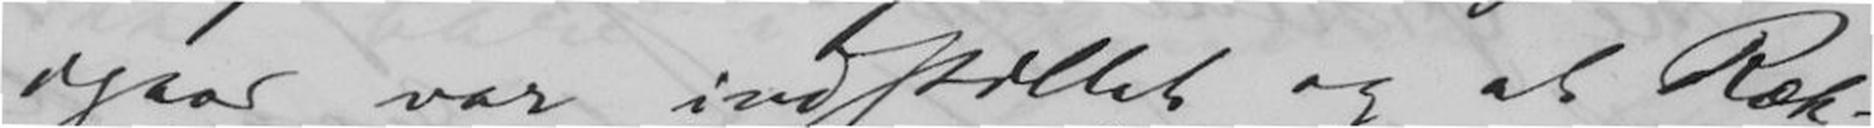igaar var indstillet og at Ræk-
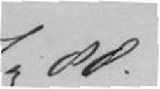20/3 88.
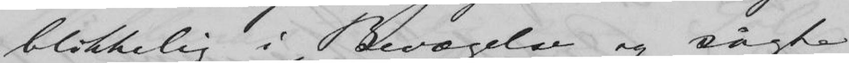blikkelig i Bevægelse og søgte
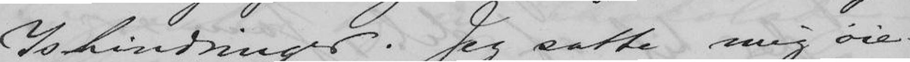Ishindringer. Jeg satte mig øie-
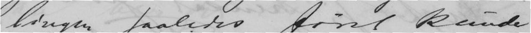lingen saaledes først kunde
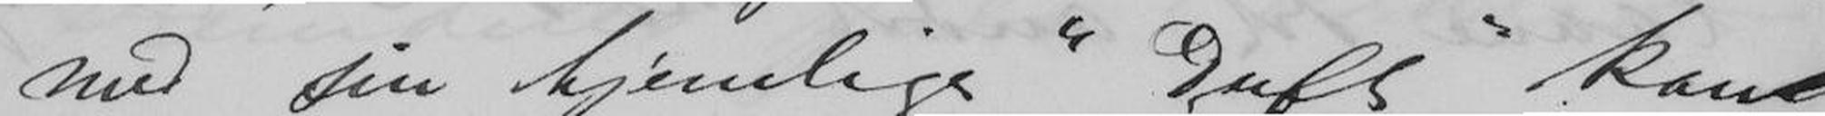med sin hjemlige "Duft" kan
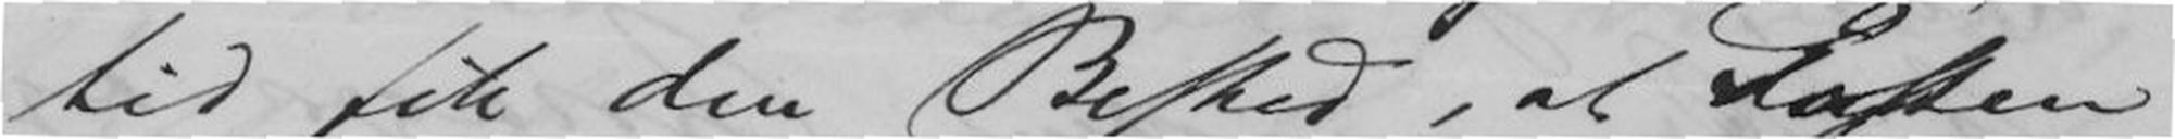tid fik den Besked, at Posten
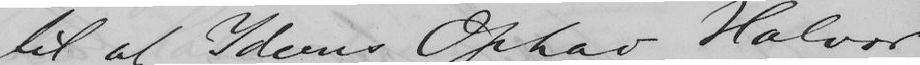til at Ideens Ophav Halvor-
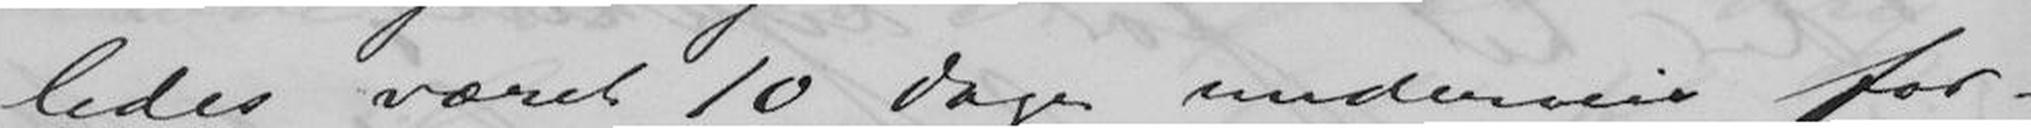ledes været 10 dage underveis for-
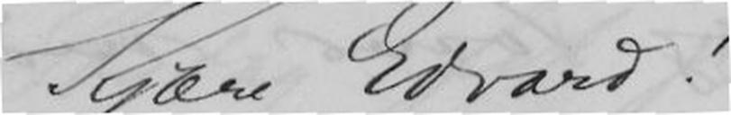Kjære Edvard!
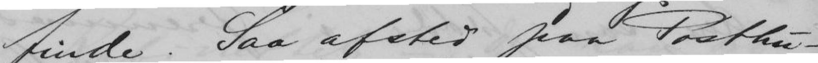finde. Saa afsted paa Posthu-
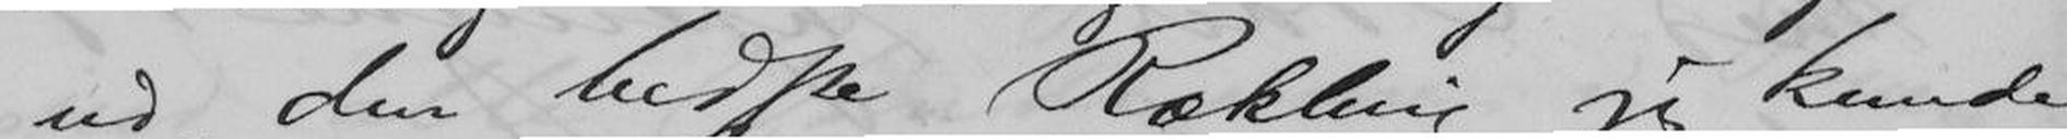ud den bedste Rækling jeg kunde
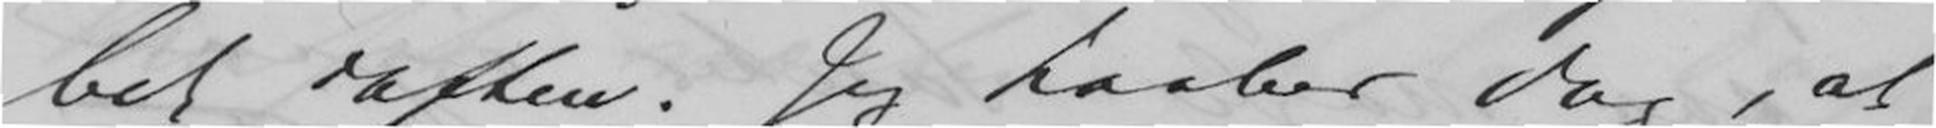bet iaften. Jeg haaber dog, at
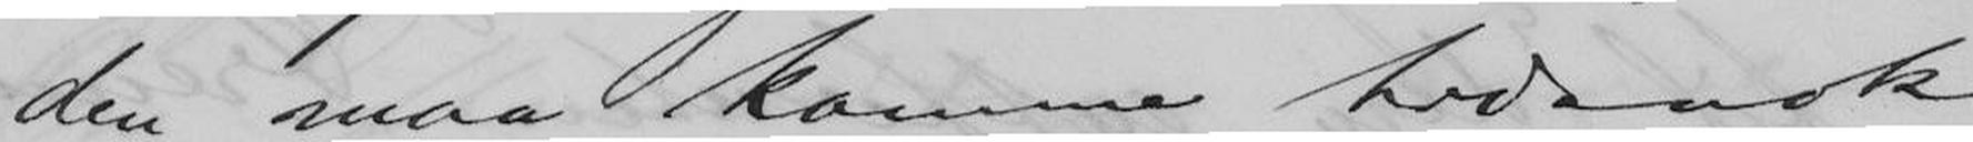den maa komme tidsnok
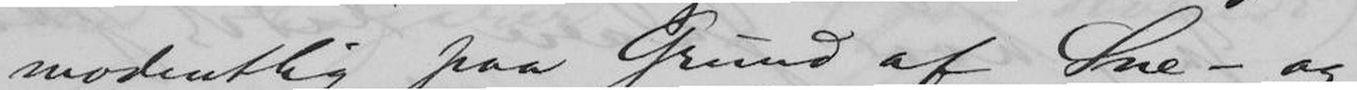modentlig paa Grund af Sne- og
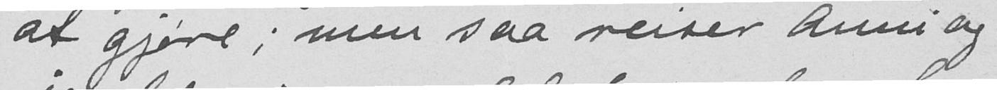at gjøre, men saa reiser Anni og
